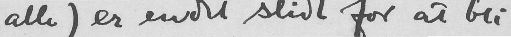alle) er endel slidt for at bli
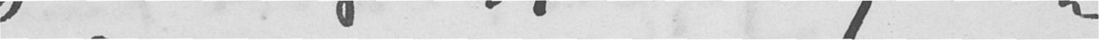ge mangler jeg samt Bjørnson
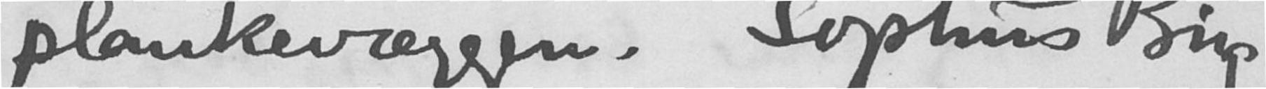plankevæggen. Sophus Bug-
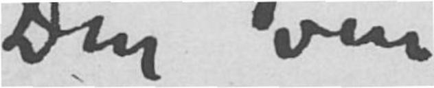Din ven
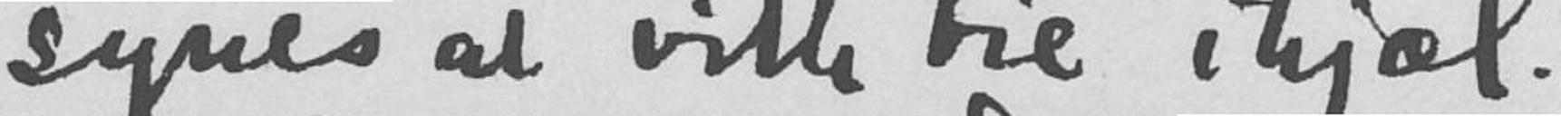synes at ville tie ihjæl.
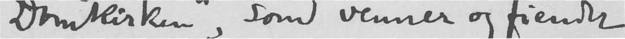"Domkirken", som venner og fiender
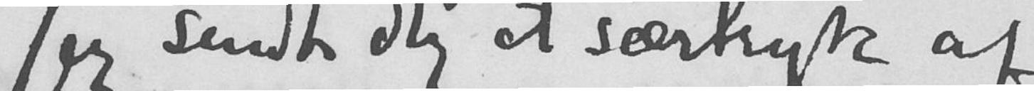Jeg sender dig et særtryk af
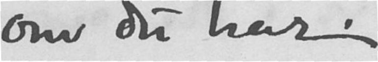om du har.
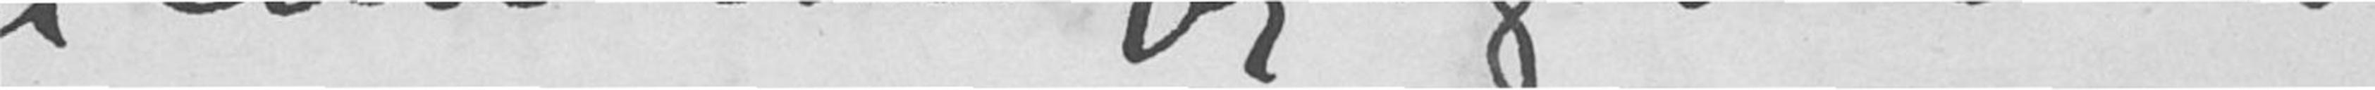"Abel" vilde jeg også ha med
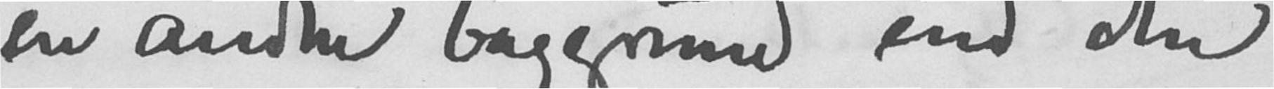en anden baggrund end den
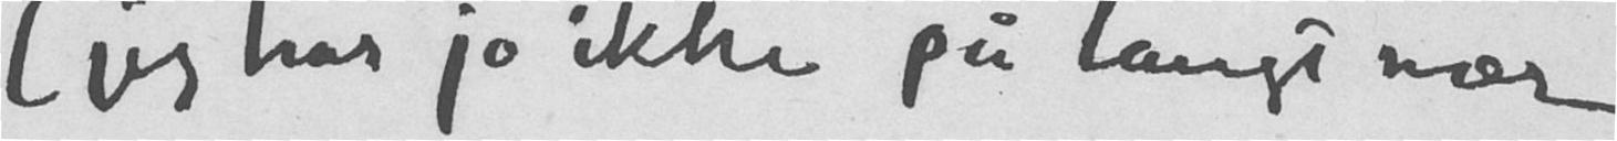(jeg har jo ikke på langt nær
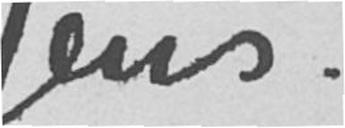Jens.
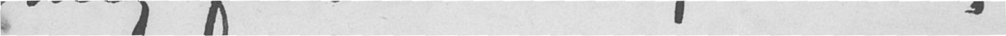mig friske exemplarer,
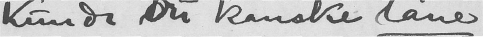Kunde Du kanske låne
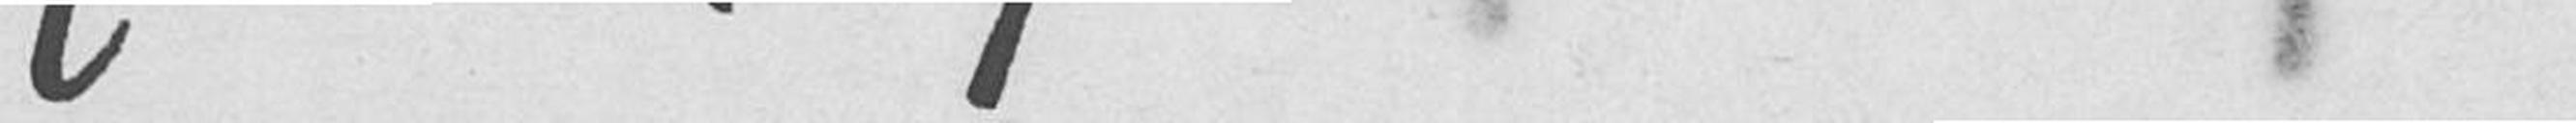gode i reproduktion.
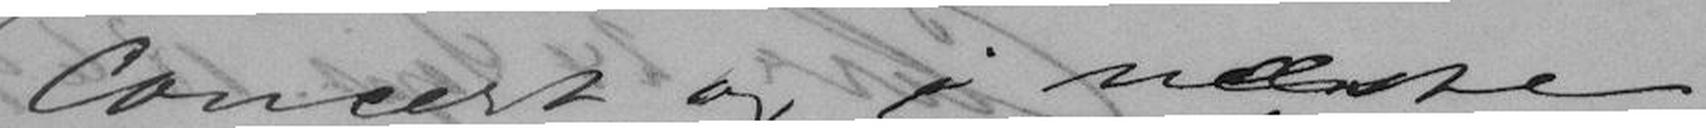Concert og i næste
